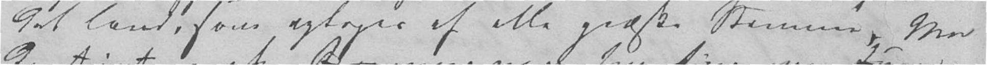det Land, som optoges af alle græske Stammer. Men
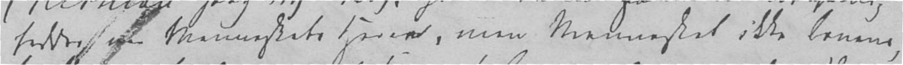hedder var Menneskets Herre, men Mennesket ikke Lovens
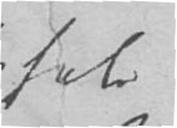hele
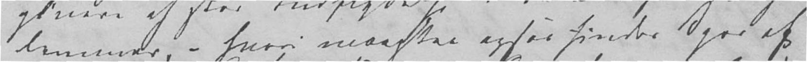dlemmer, – hvori maaskee ogsaa findes Spor af
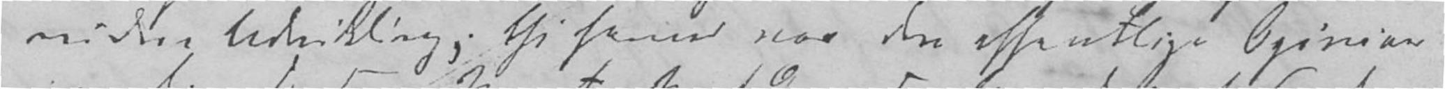videre Udvikling; thi senere var den offentlige Opinion
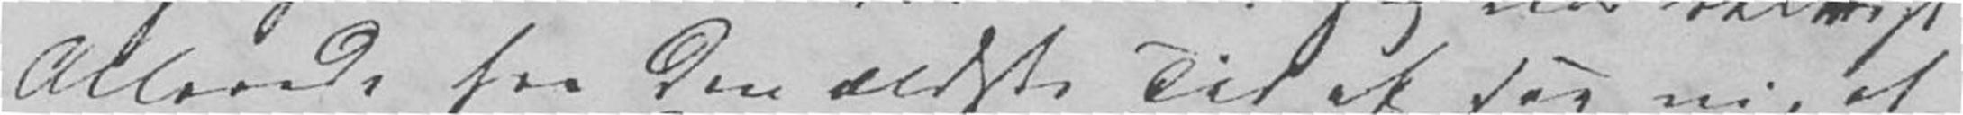Allerede fra den ældste Tid af see vi, at
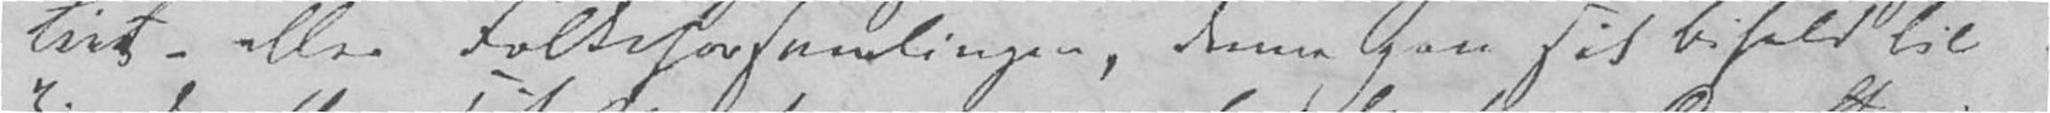tiet – eller Folkeforsamlingen, denne gav sit Bifald til
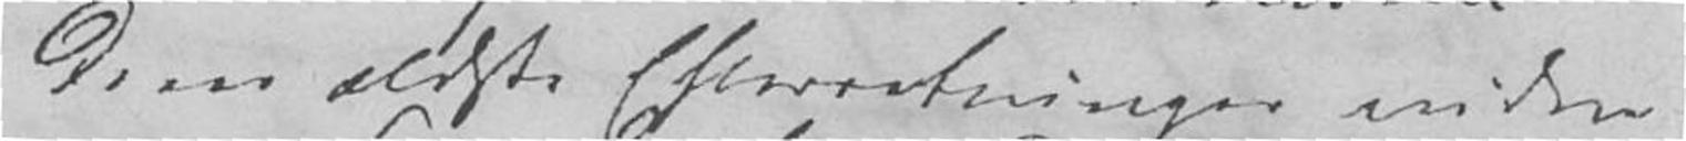Deres ældste Efterretninger vidner
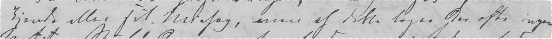Kjende eller sit Lidetog, men af dette toges der ofte ingen
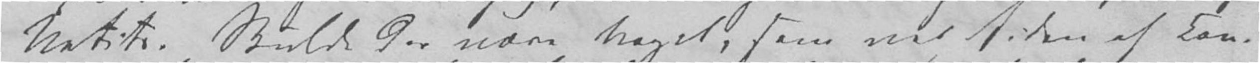Notits. Skulde der være noget, som ved Siden af Kon-
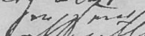som X
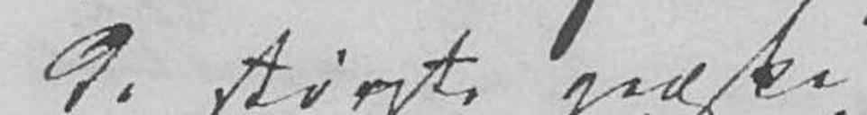de største græske
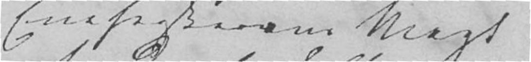Eneherskerens Magt
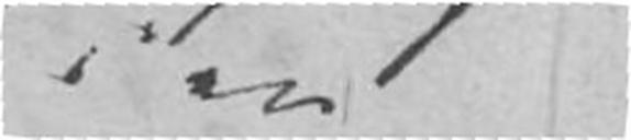i en
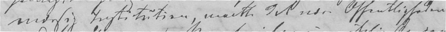mæssig Institution, maatte det være Offentligheden
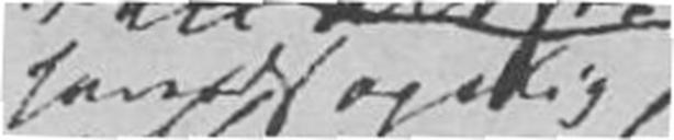hovedsagelig
